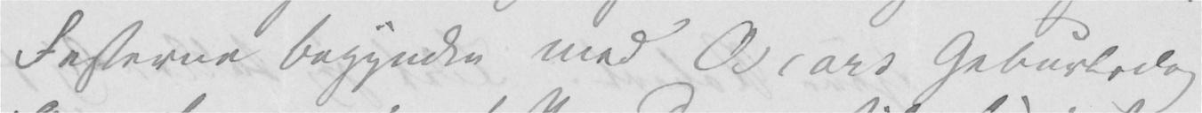Festerne begyndte med Oscars Geburtsdag
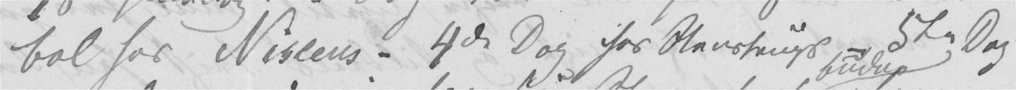bal hos Nissens – 4de Dag hos Stenstrups – 5te Dag
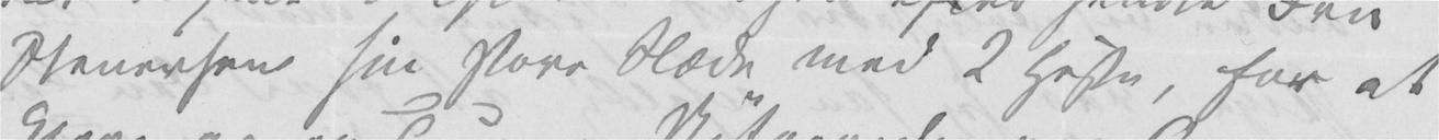Stenersen sin store Slæde med 2 Heste, for at
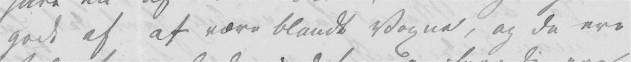godt af at være blandt Voxne, og de ere
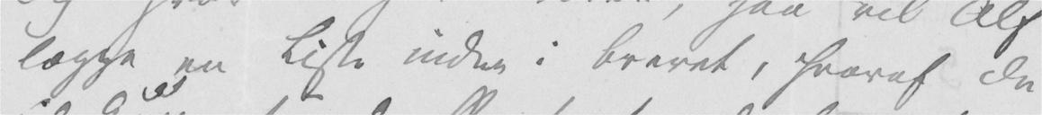lægge en Liste inden i Brevet, hvoraf Du
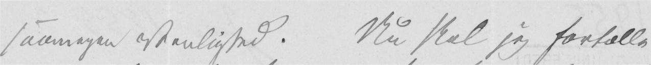saamegen Venlighed. Nu skal jeg fortælle
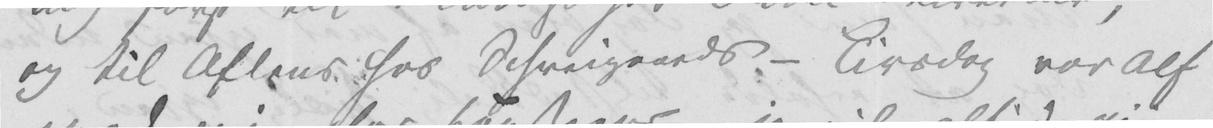og til Aftens hos Schweigaards. – Tirsdag var Alf
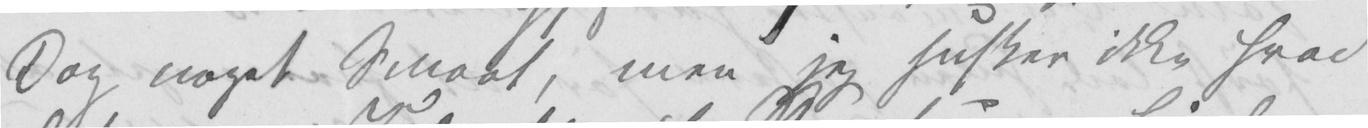Dag noget Smaat, men jeg husker ikke hvad
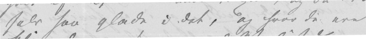selv saa glade i det, og hvor de ere
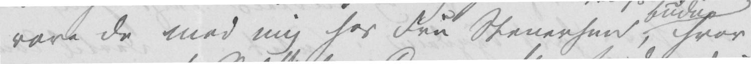vare de med mig hos Fru Stenersen, hvor
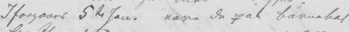Iforgaars 5te Jan. vare de paa Børnebal
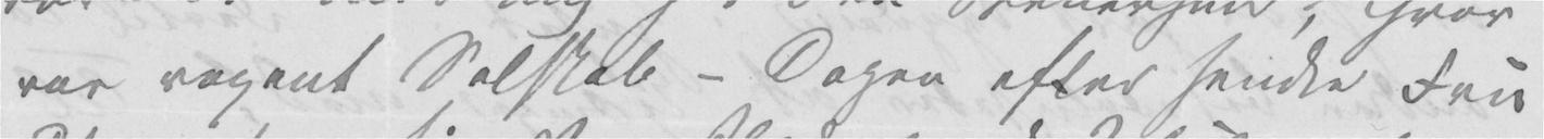var voxent Selskab – Dagen efter sendte Fru
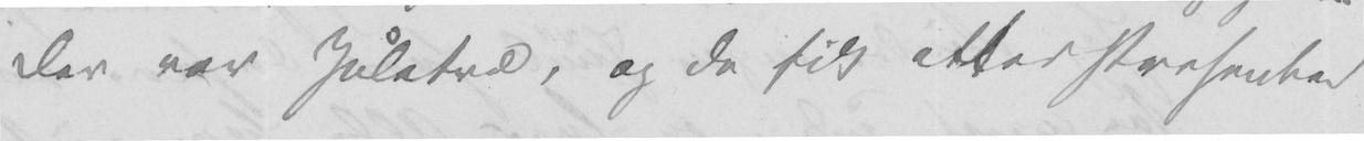Der var Juletræ, og de fik atter Presenter
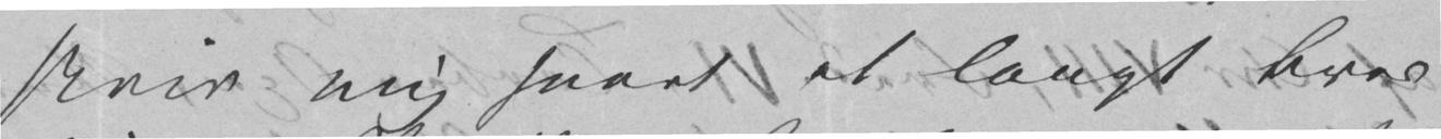skriv mig snart et langt Brev
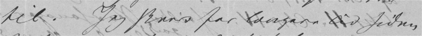til. Jeg skrev for længere Tid siden
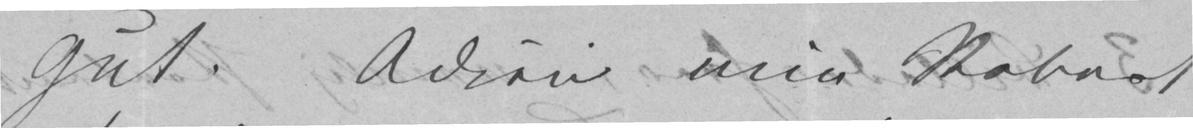Gut. Adieu min Robert
